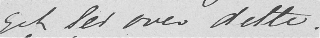get lei over dette.
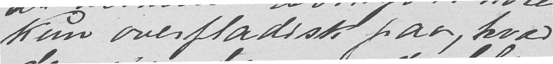kun overfladisk paa, hvad
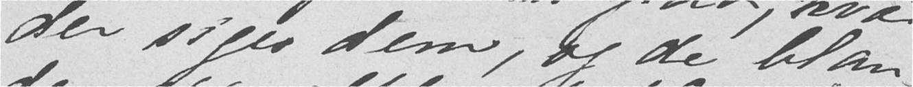der siges dem, og de blan-
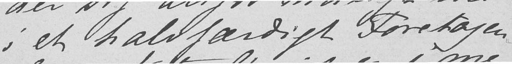i et halvfærdigt Foretagen-
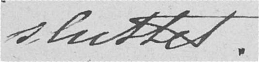sluttet.
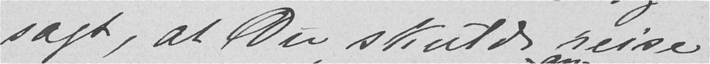sagt, at Du skulde reise
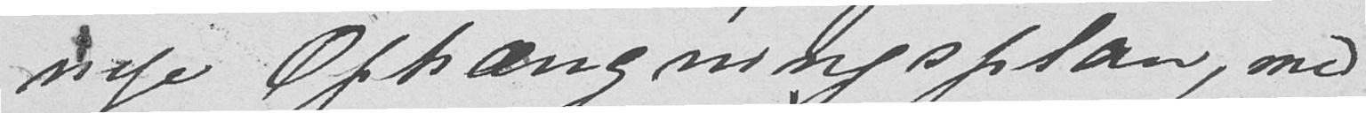nye Ophængningsplan, med
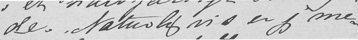de. Naturligvis er jeg me-
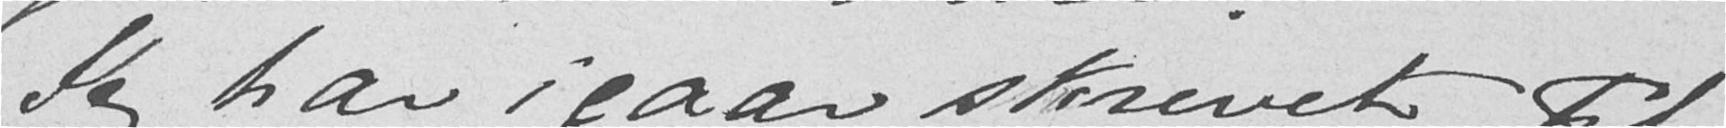Jeg har igaar skrevet til
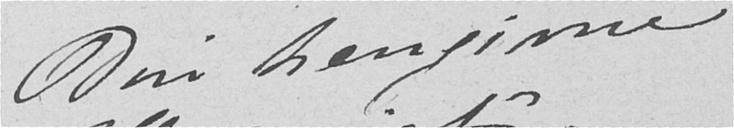Din hengivne
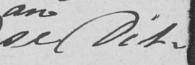se Dit
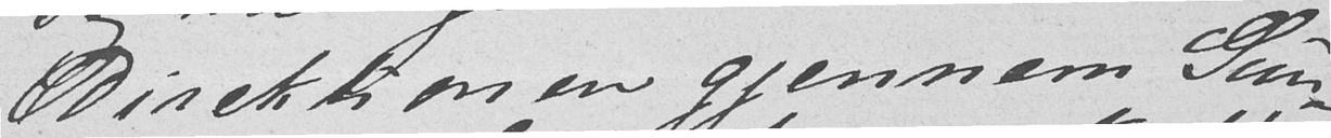Direktionen gjennem Gun-
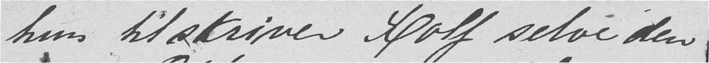hun tilskriver Rolf selve den
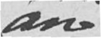an-
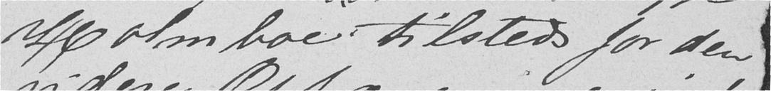Holmboe tilstede for den
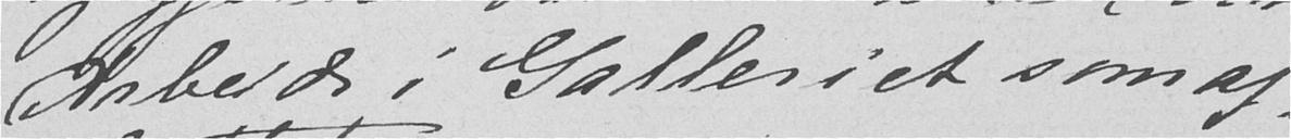Arbeide i Galleriet som af-
